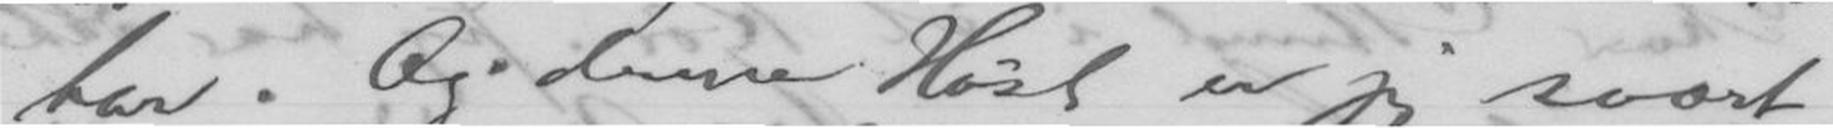har. Og denne Høst er jeg svært
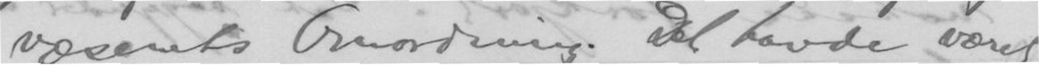væsenets Omordning. Det havde været
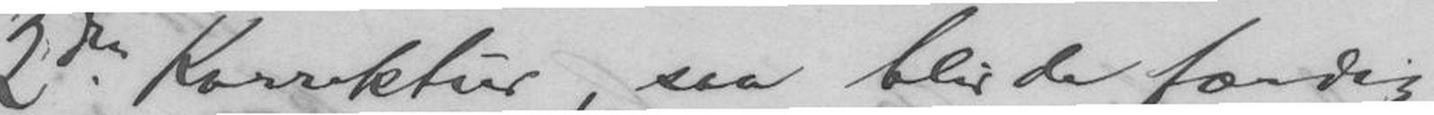2den Korrektur, saa blir de færdig
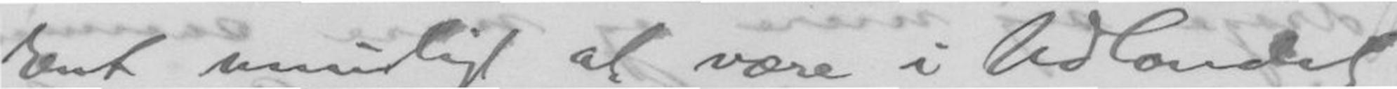rent umuligt at være i Udlandet
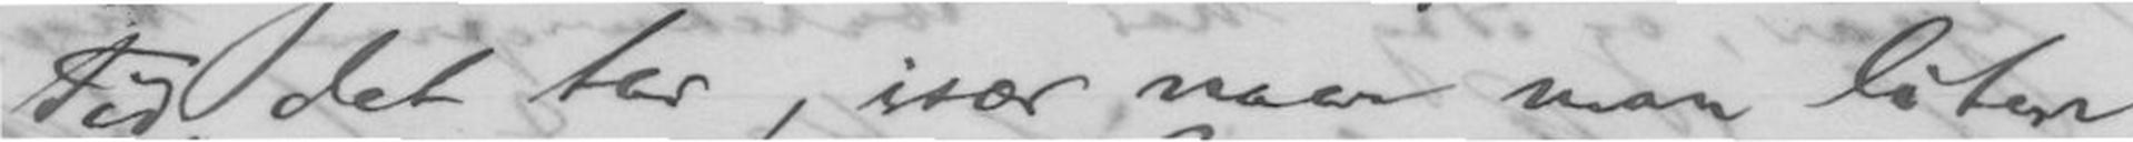Tid det tar, især naar man liten
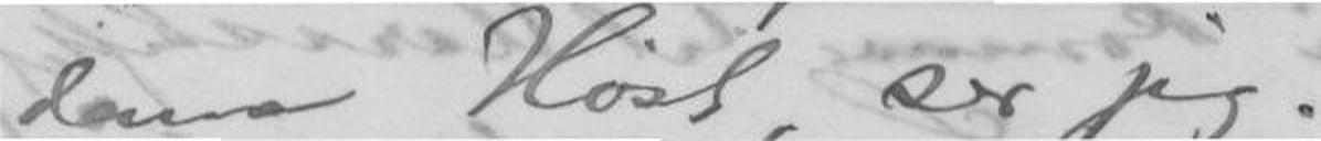denne Høst, ser jeg.
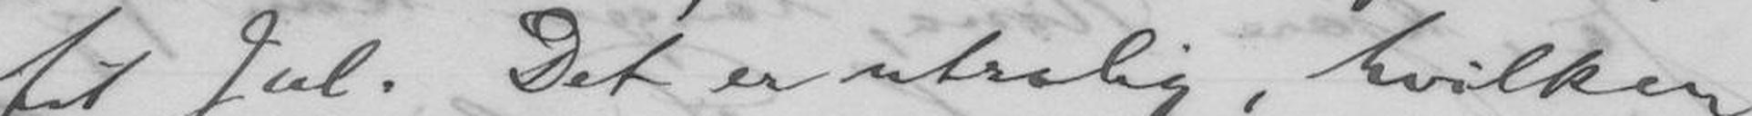til Jul. Det er utrolig, hvilken
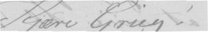Kjære Grieg!
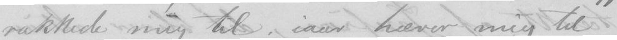rakkede mig til, iaar hæver mig til
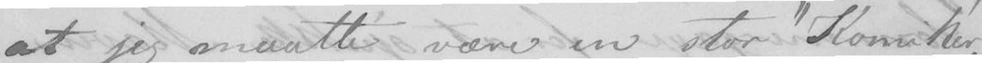at jeg maatte være en stor "Komiker
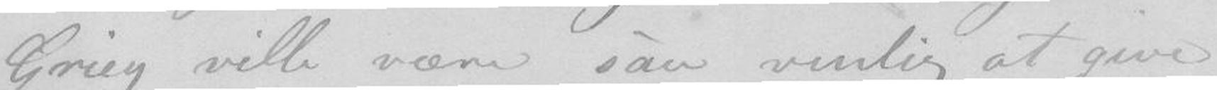Grieg ville være saa venlig at give
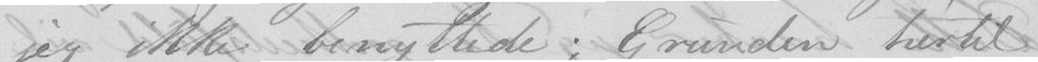jeg ikke benyttede; Grunden hertil
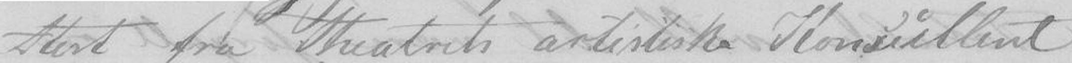Atest fra Theatrets artistiske Konsullent
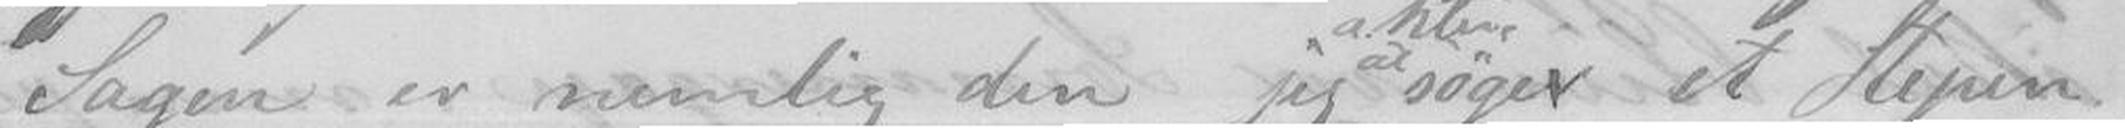Sagen er nemlig den jeg søge et Stipen-
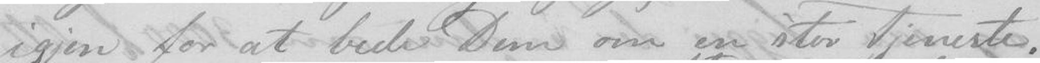igjen for at bede Dem om en stor Tjeneste.
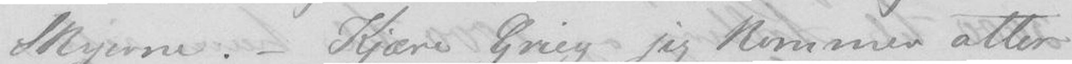Skyerne. - Kjære Grieg jeg kommer atter
